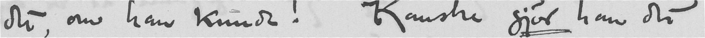det, om han kunde! Kanske gjør han det
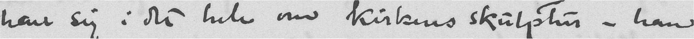han sig i det hele om kirkens skulptur - han
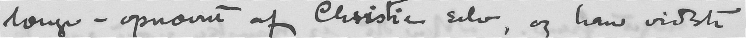længe - opnævnt af Christie selv, og han vidste
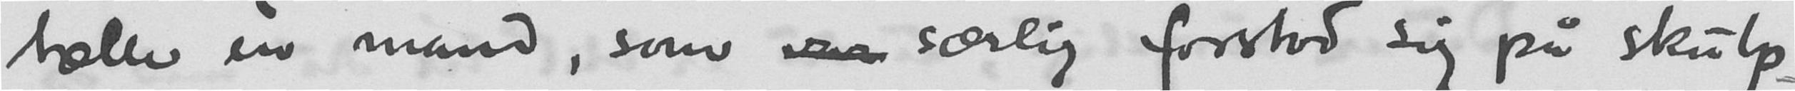tælle en mand, som var særlig forstod sig på skulp-
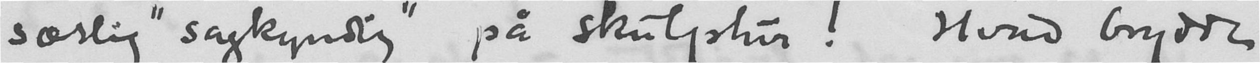særlig "sagkyndig" på skulptur! Hvad brydde
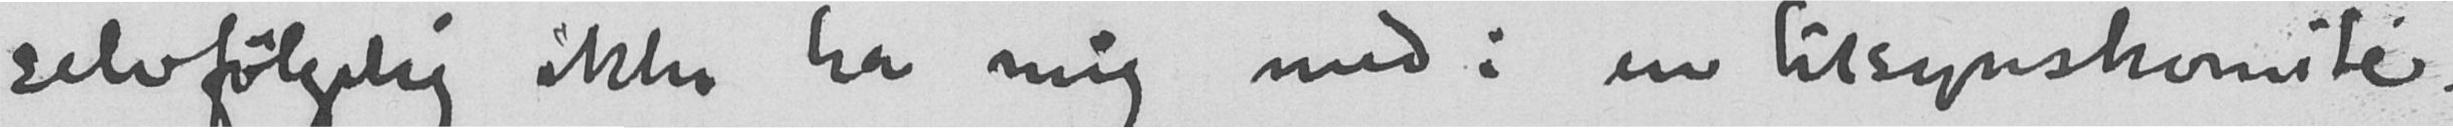selvfølgelig ikke ha mig med i en tilsynskomité.
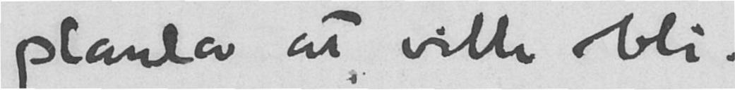planla at ville bli.
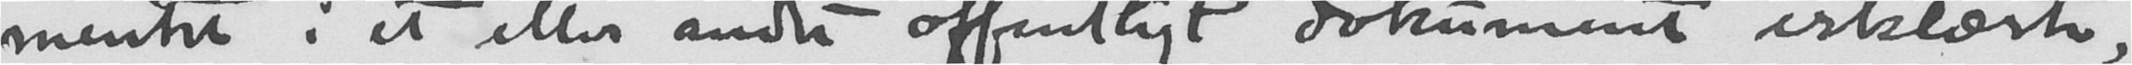mentet i et eller andet offentligt dokument erklærte,
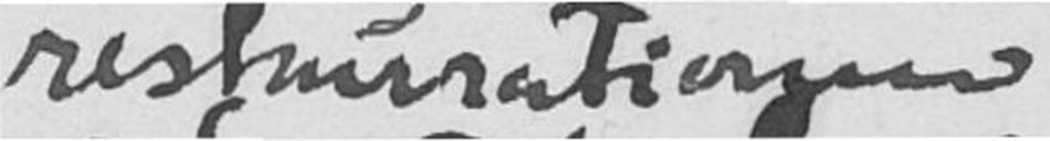restaurationen
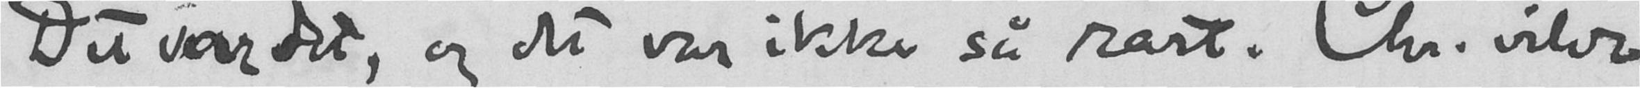Det var det, og det var ikke så rart. Chr. vilde
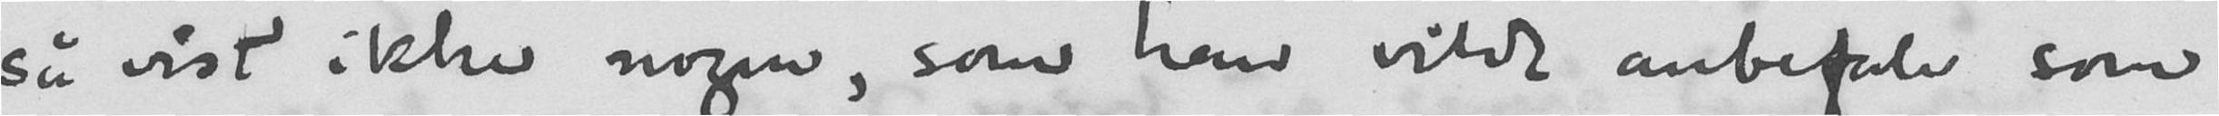så vist ikke nogen, som han vilde anbefale som
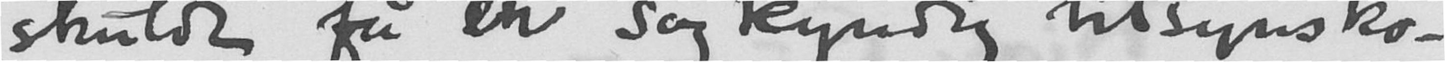skulde få en sagkyndig tilsynsko-
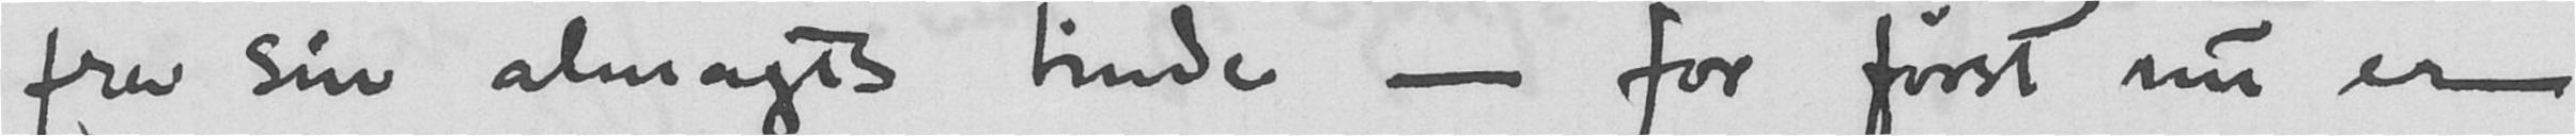fra sin almagts tinde - for først nu er
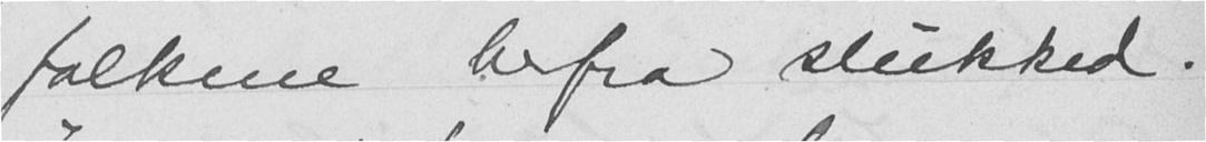folkene herfra slukked.
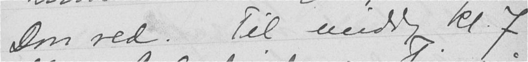Don red. Til middag kl. 7
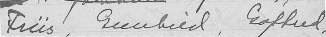Friis, Gunhild, Gotfred,
